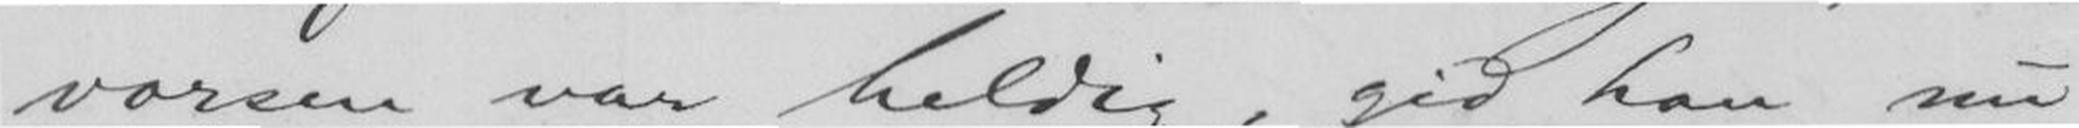vorsen var heldig, gid han nu
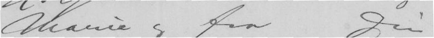Marie og fra Din
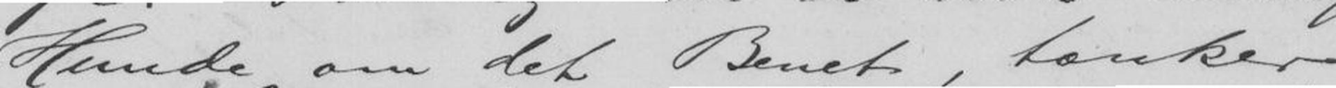Hunde om det Benet, tænker
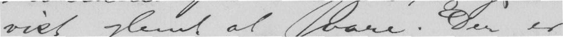vist glemt at svare. Der er
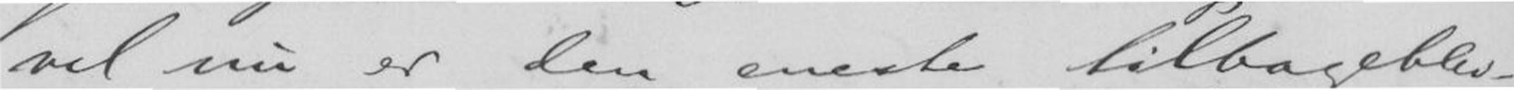vel nu er eneste tilbageblev-
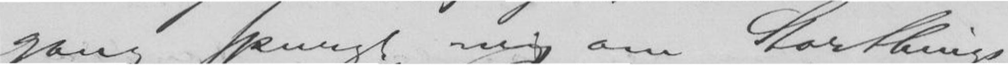gang spurgt mig om Storthings-
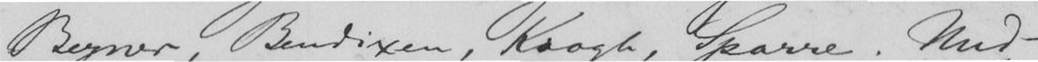Begner, Bendixen, Krogh, Sparre. Und-
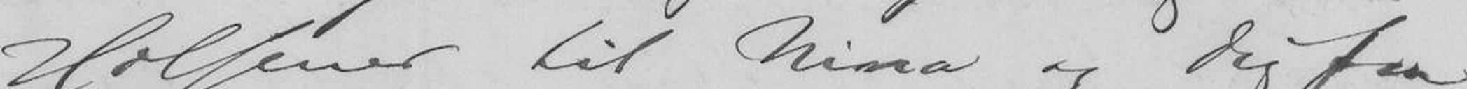Hilsener til Nina og Dig fra
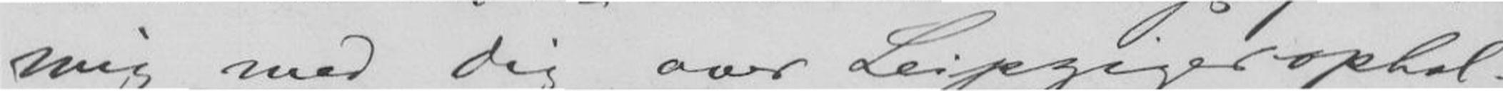mig med Dig over Leipzigerophol-
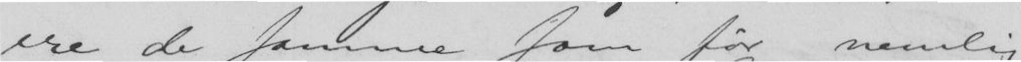ere de samme som før nemlig
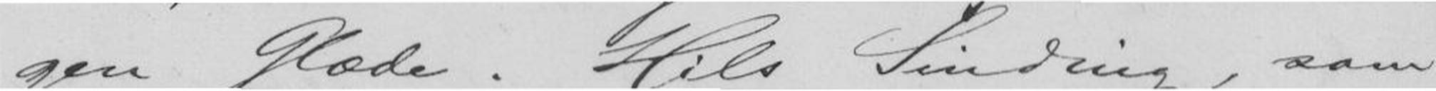gen Glæde. Hils Sinding, som
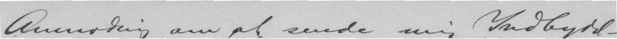Anmodning om at sende mig Indbydel-
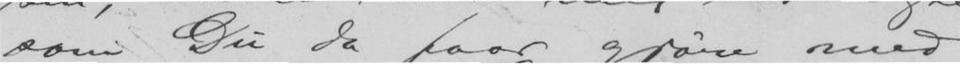som Du da faar gjøre med
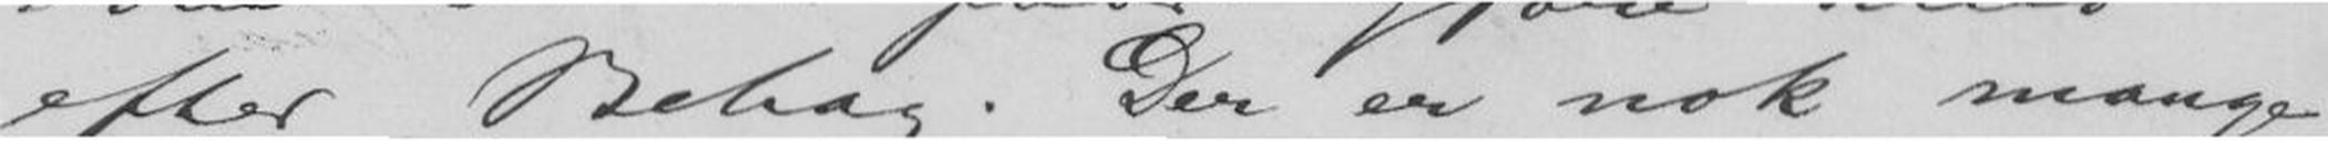efter Behag. Der er nok mange
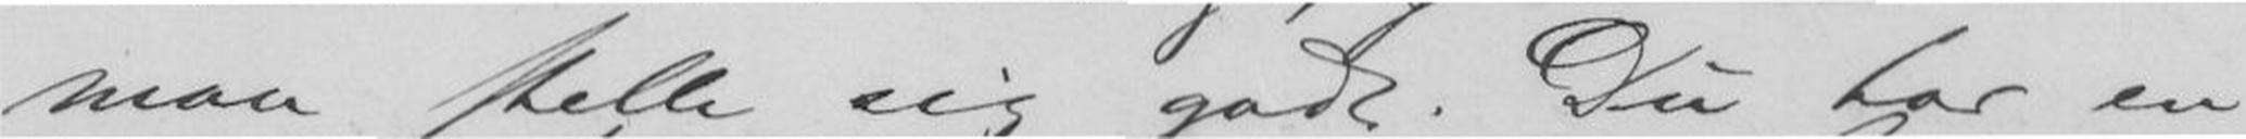maa stelle sig godt. Du har en
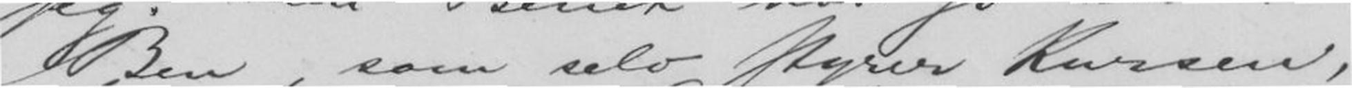Ben, som selv styrer Kursen,
